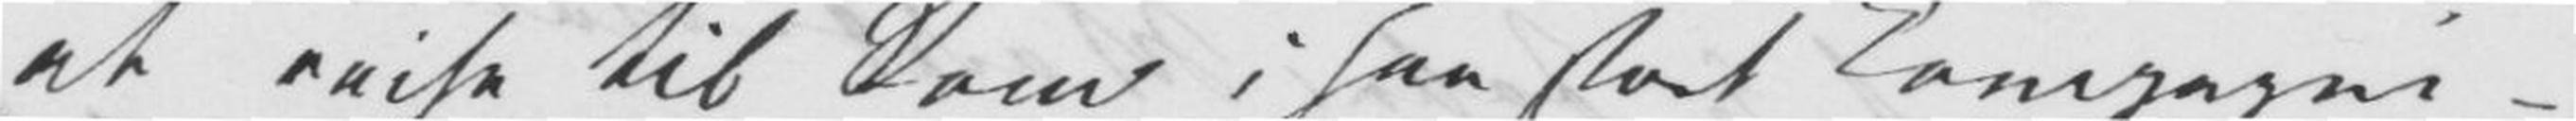at reise til Rom i saa stort Kompagni –
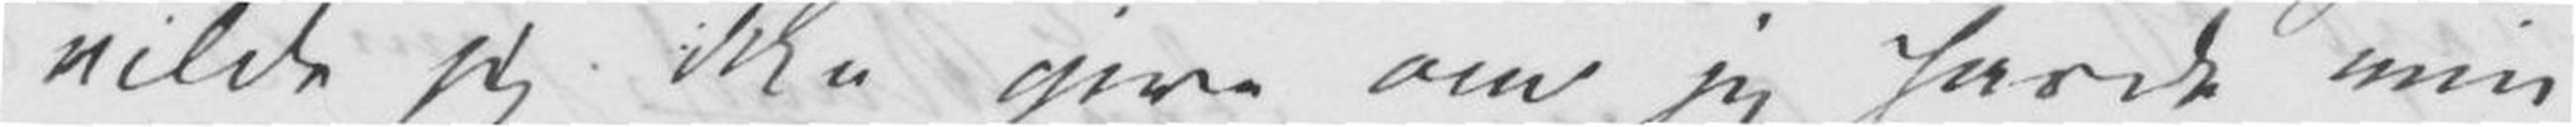vilde jeg ikke give om jeg havde min
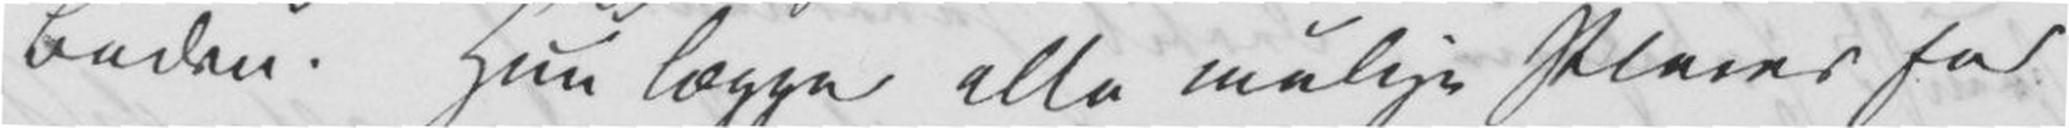Baden. Hun lægger alle mulige Planer for
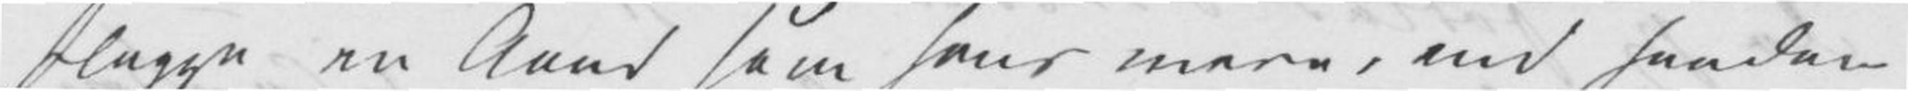stegge en Aand som hans mere, end saadan
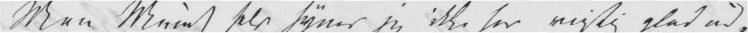Men Munch selv synes jeg ikke ser rigtig glad ud,
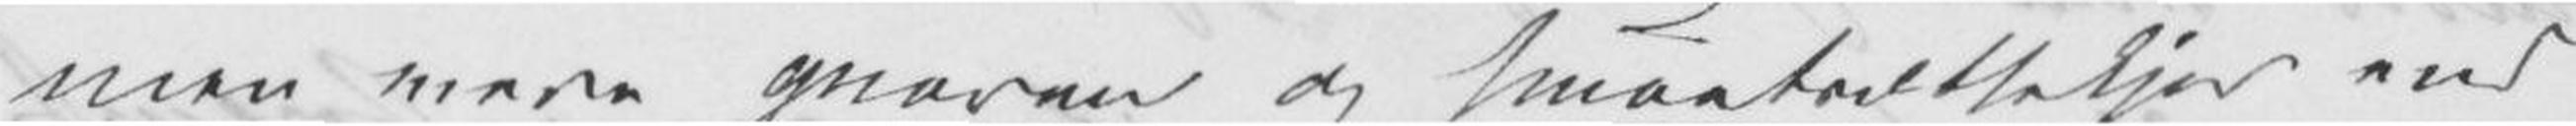men mere gnaven og smaatrættekjer end
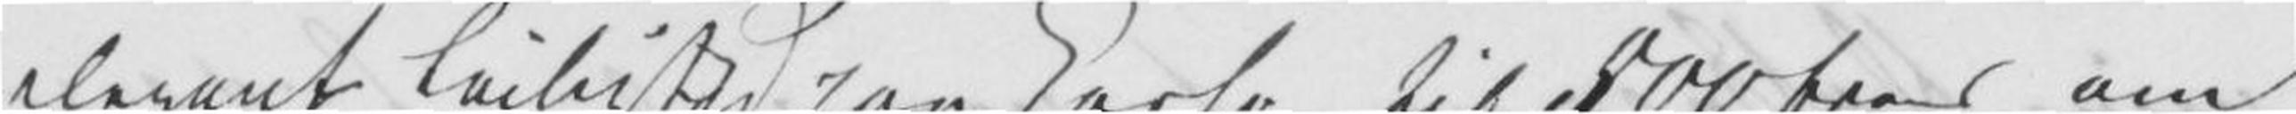elegant Leilighed paa Corso til 500 franc om
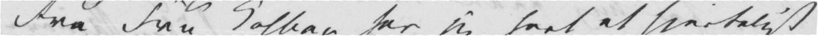Fra Fru Colban har jeg havt et hjerteligt
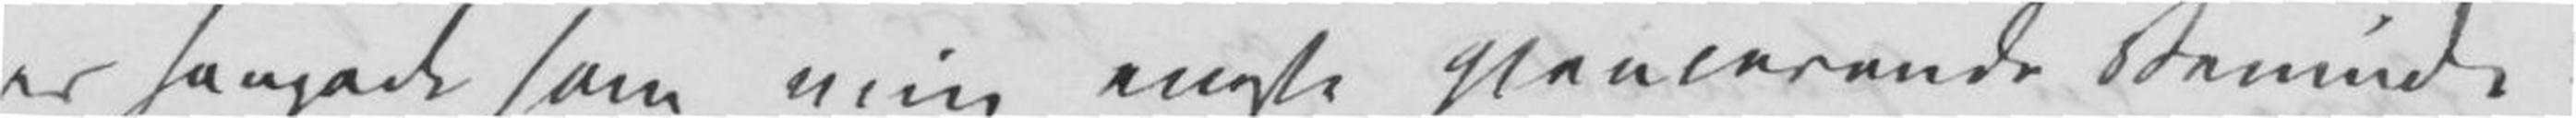er saagodt som min eneste gjenlevende Veninde.
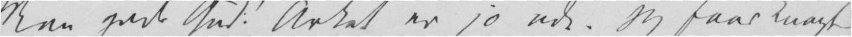Men gode Gud! Arket er jo ude! Jeg faar knapt
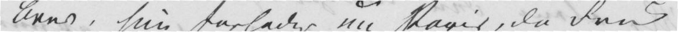Brev, hun forlader nu Paris, da Fru
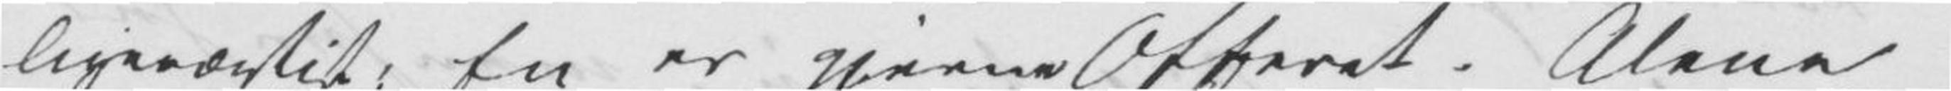ligevægtigt; En er gjerne Offeret.[)] Alene
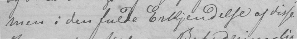men i den fulde Erkjendelse af disse
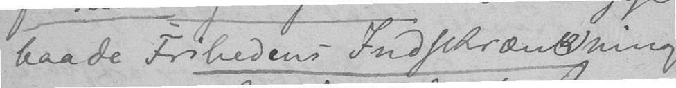baade Frihedens Indskrænkning
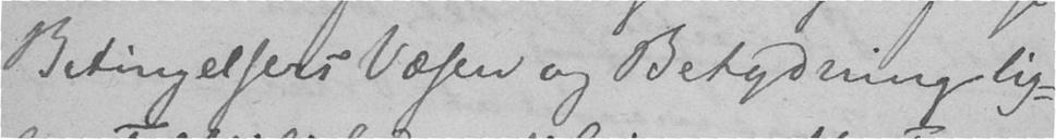Betingelsers Væsen og Betydning lig-
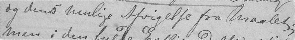og dens mulige Afvigelse fra Maalet;
